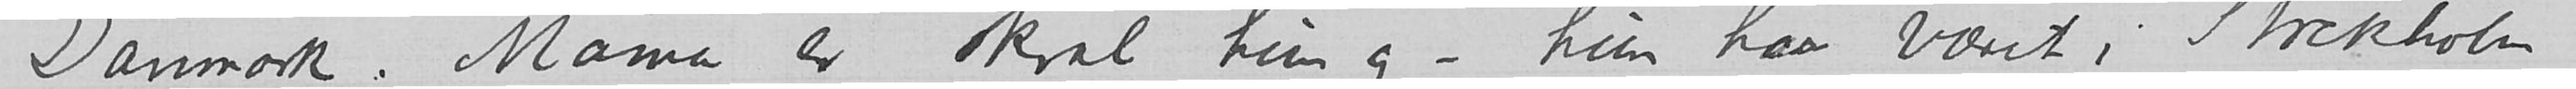Danmark. Mama er skral hun og – hun har været i Stockholm
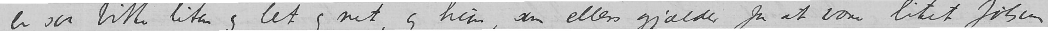er saa bitte liten og let og net, og hun, som ellers gjælder for at være litet følsom
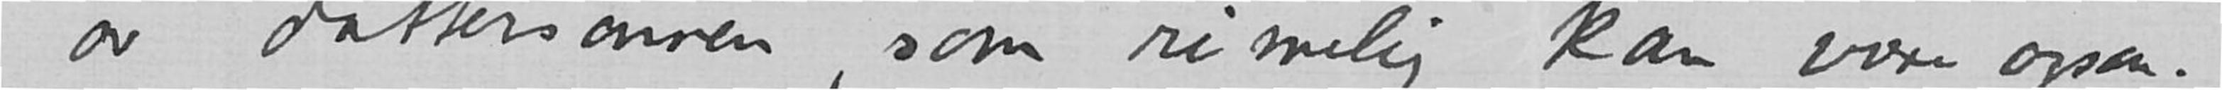av dattersønnen, som rimelig kan være ogsaa.
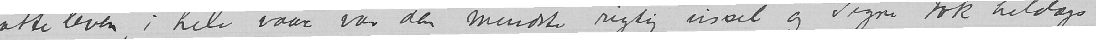natteleven, i hele vaar var den mindste rigtig ussel og Signe tok heldags
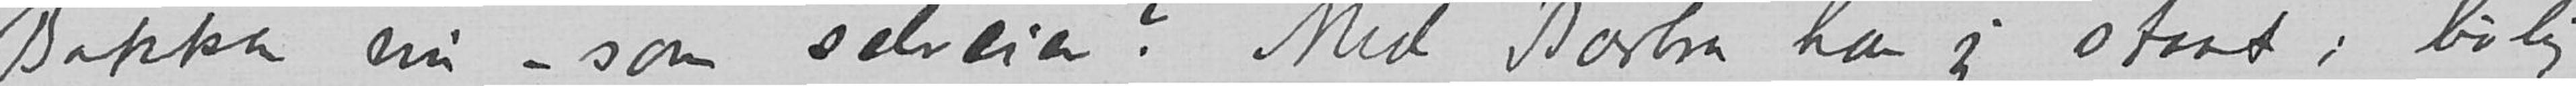Bakka nu – som selveier? Med Barbra har jeg staat i livlig
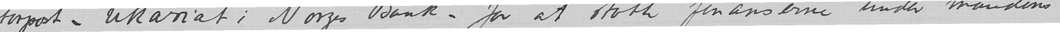kontorpost-vikariat i Norges Bank – for at støtte finanserne under mandens
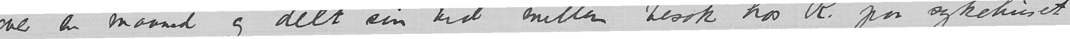over en maaned og delt sin tid mellem besøk hos R. paa sykehuset
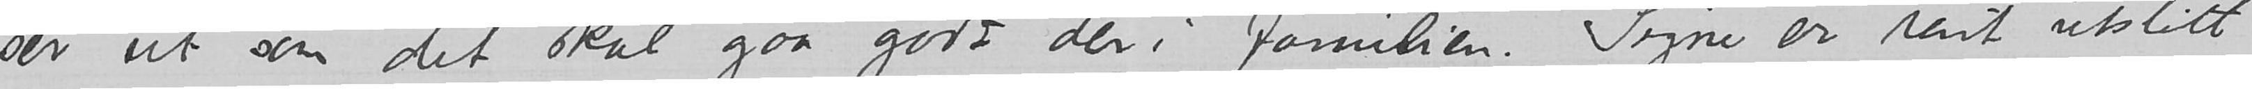ser ut som det skal gaa godt der i familien. Signe er rent utslitt
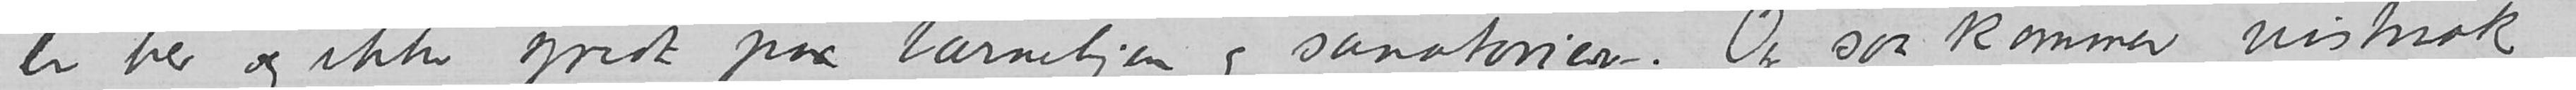er her og ikke spredt paa barnehjem og sanatorier. Og saa kommer vistnok
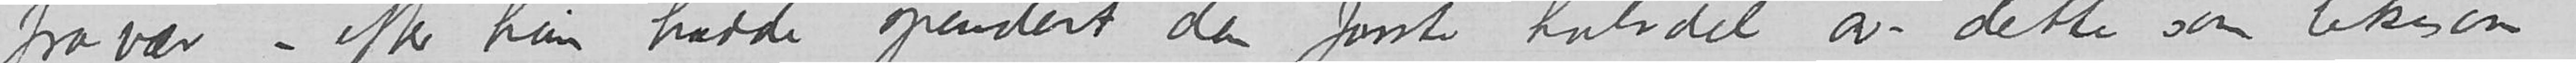fravær – efter hun hadde spandert den første halvdel av dette som likesom
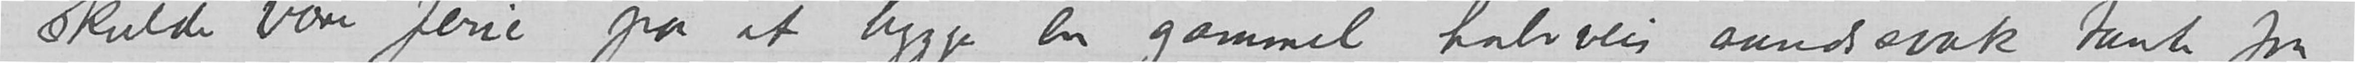skulde være ferie paa at hygge en gammel, halvveis aandsvak tante fra
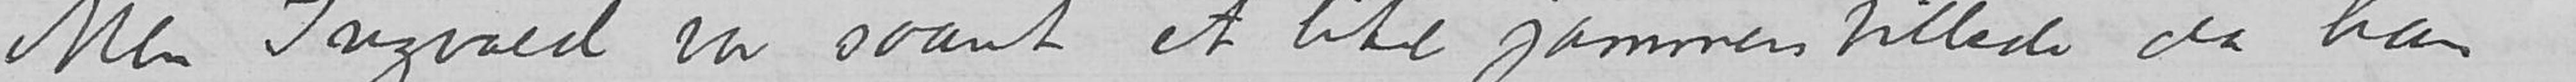Men Ingvald var saant et lite jammersbillede da han
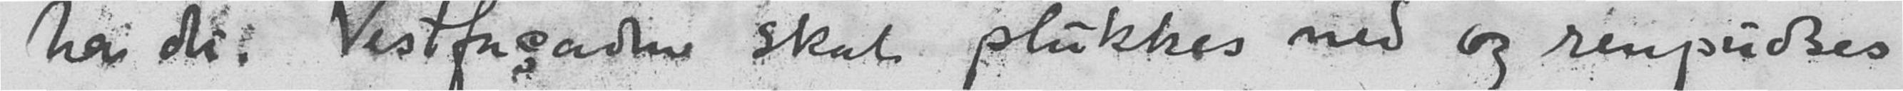ha det. Vestfacaden skal plukkes ned og renpudses
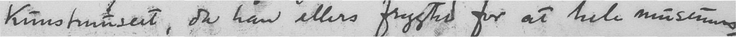Kunstmuseet, da han ellers frygted for at hele museums-
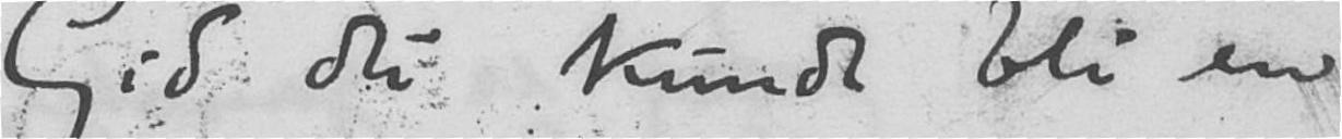Gid du kunde bli en
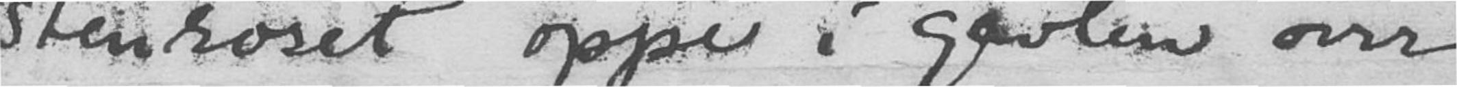stenroset oppe i gavlen over
